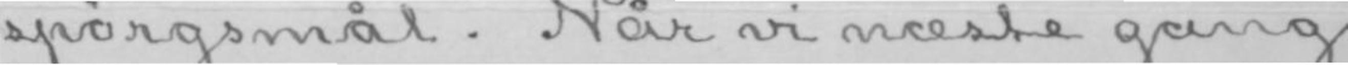spørgsmål. Når vi næste gang
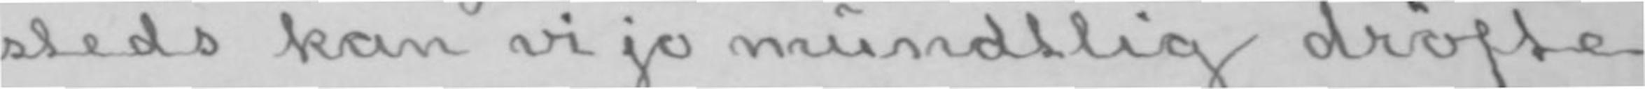steds kan vi jo mundtlig drøfte
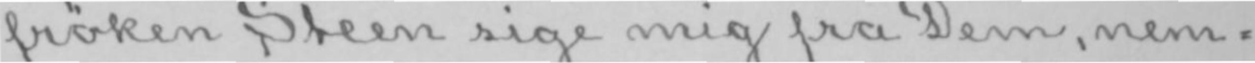frøken Steen sige mig fra Dem, nem-
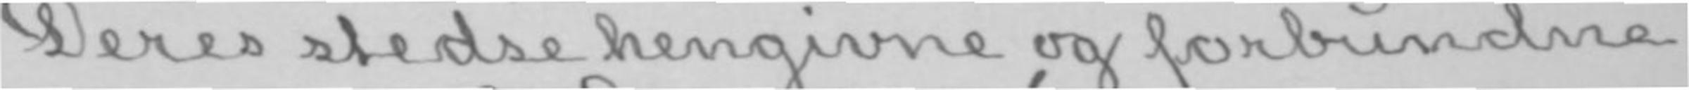Deres stedse hengivne og forbundne
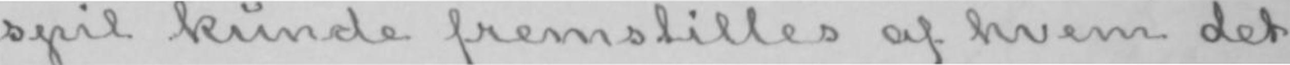spil kunde fremstilles af hvem det
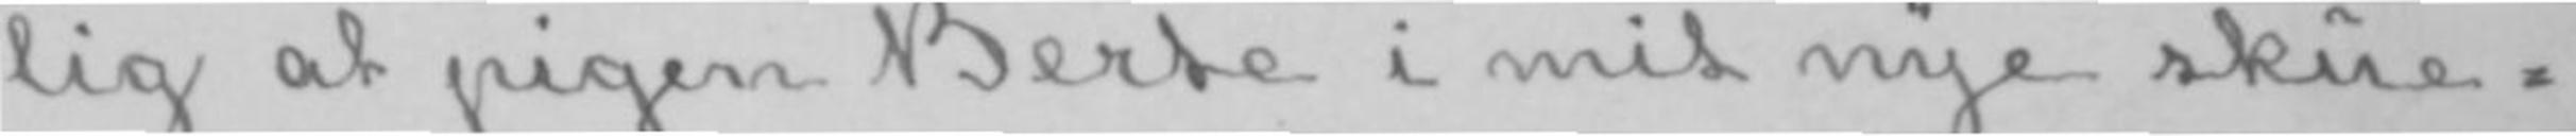lig at pigen Berte i mit nye skue-
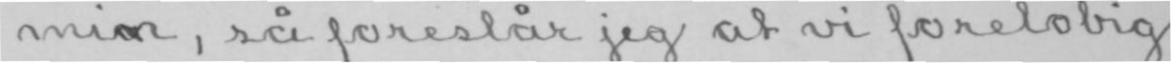min, så foreslår jeg at vi foreløbig
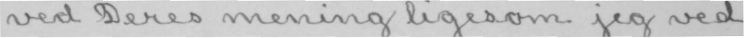ved Deres mening ligesom jeg ved
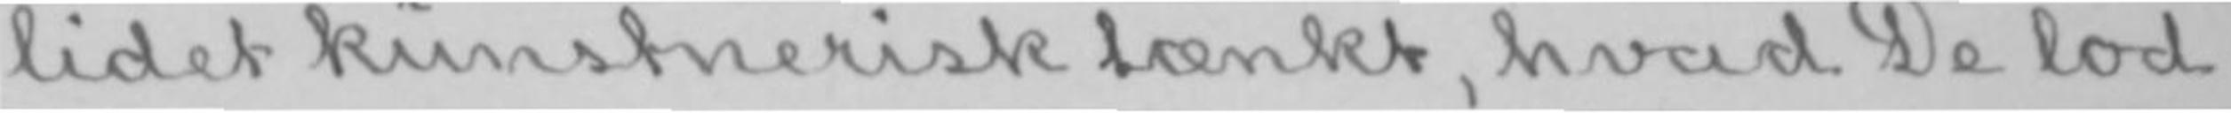lidet kunstnerisk tænkt, hvad De lod
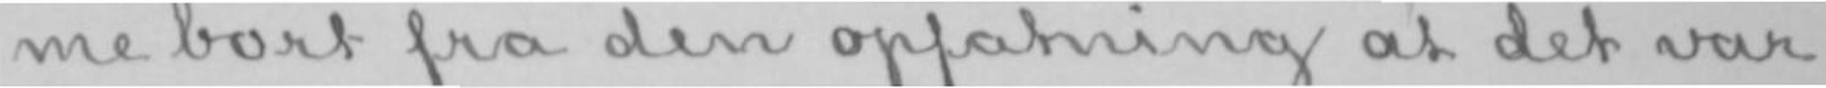me bort fra den opfatning at det var
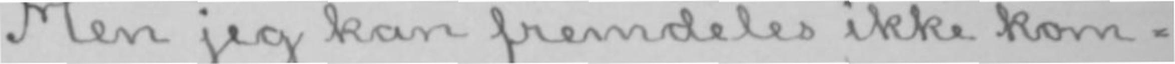Men jeg kan fremdeles ikke kom-
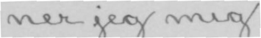ner jeg mig
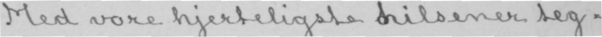Med vore hjerteligste hilsener teg-
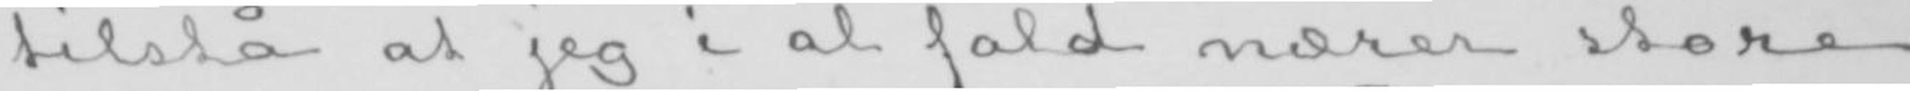tilstå at jeg i al fald nærer store
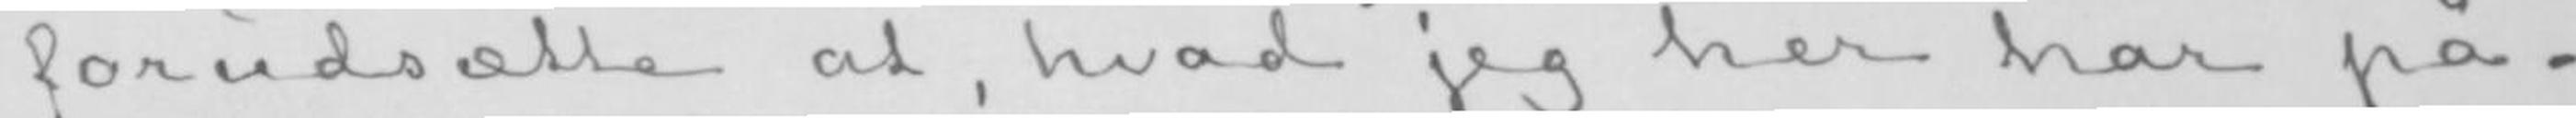forudsætte at, hvad jeg her har på-
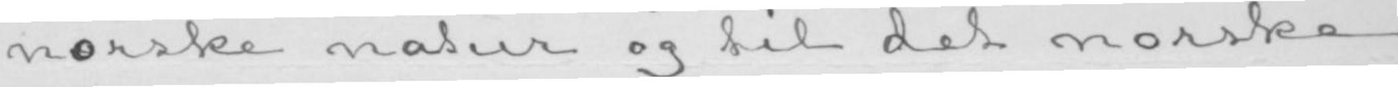norske natur og til det norske
